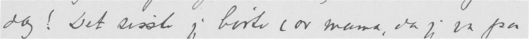dag! Det sisste jeg hørte (av mama, da jeg var paa
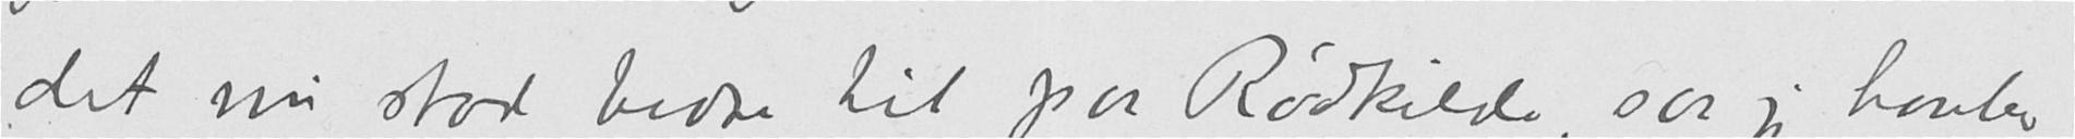det nu stod bedre til paa Rødkilde, saa jeg haaber
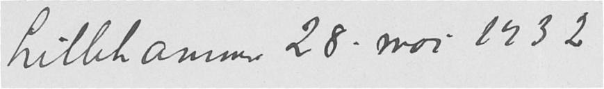Lillehammer 28. mai 1932
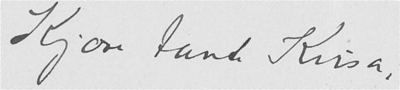Kjære tante Kirsa,
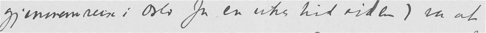gjennemreise i Oslo for en ukes tid siden) var at
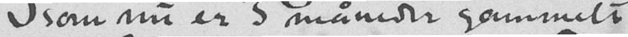som nu er 3 måneder gammelt
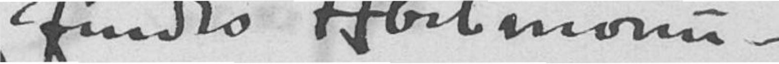findes Abelmonu-
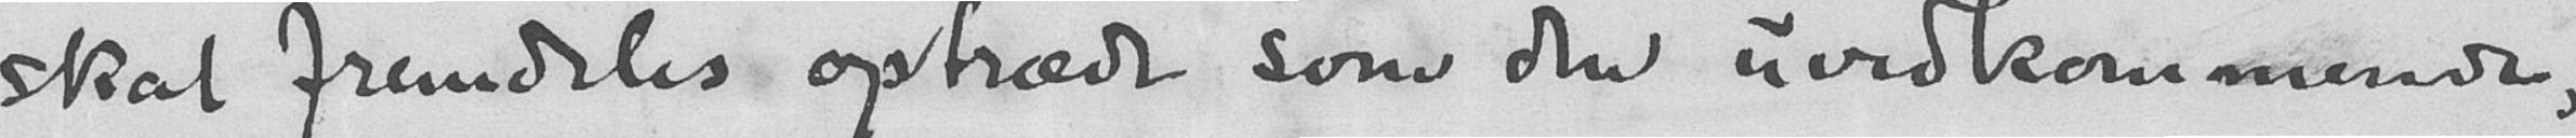skal fremdeles optræde som den uvedkommende,
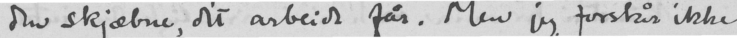den skjæbne, dit arbeide får. Men jeg forstår ikke
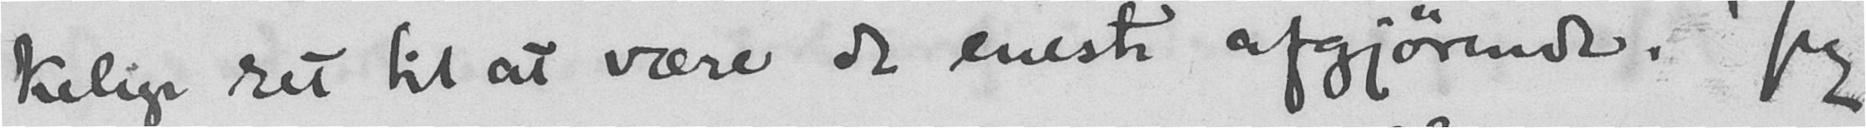kelige ret til at være de eneste afgjørende. Jeg
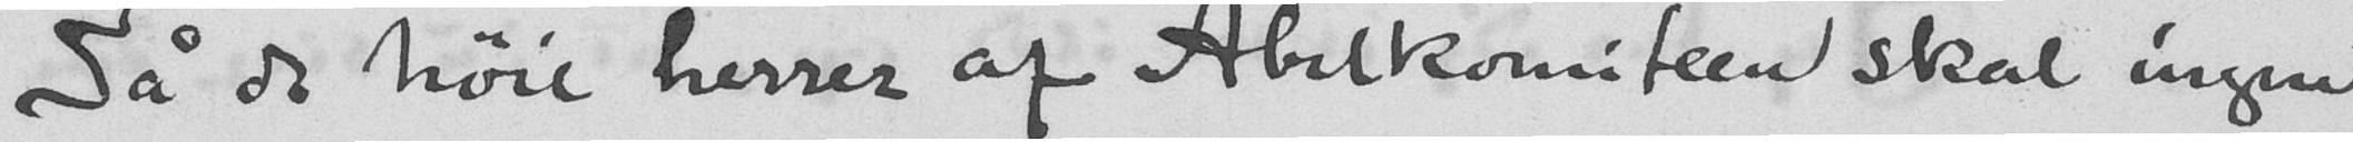Så de høie herrer af Abelkomiteen skal ingen
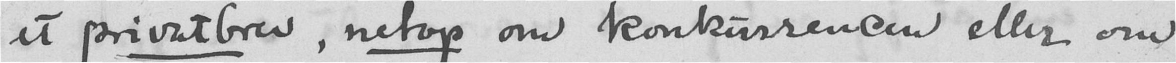et privatbrev, netop om konkurrensen eller om
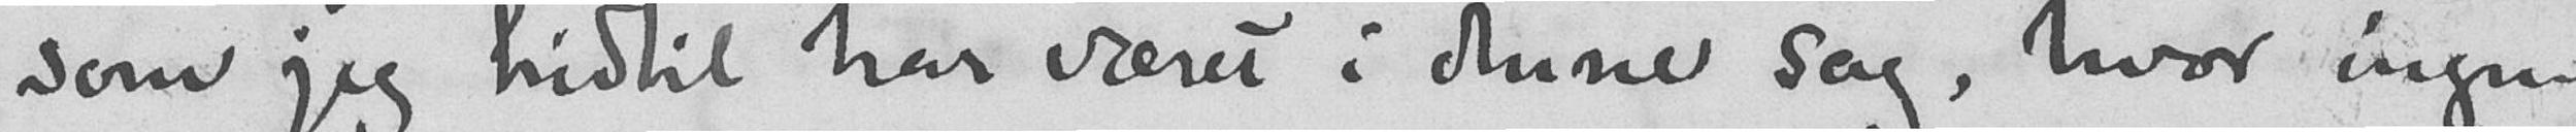som jeg hidtil har været i denne sag, hvor ingen
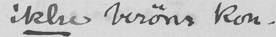ikke berører kon-
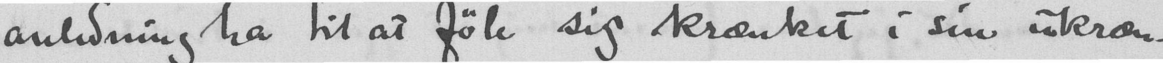anledning ha til at føle sig krænket i sin ukræn-
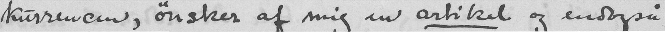kurrencen, ønsker af mig en artikel og endogså
